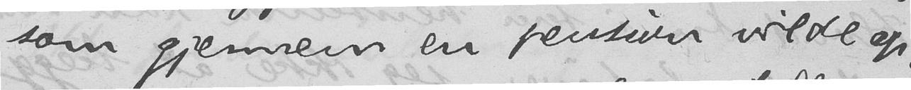som gjennem en pension vilde op-
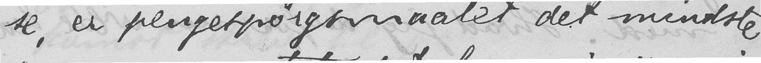se, er pengespørgsmaalet det mindste
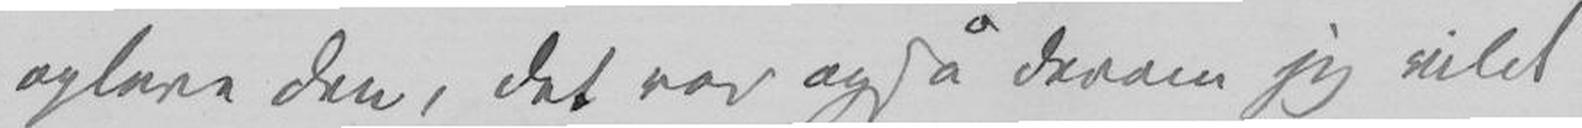opleve den, det var også derom jeg vilde
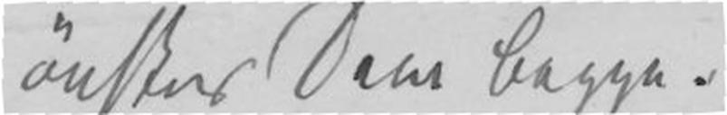ønskes Dere begge.
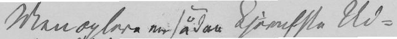Vanoplere er sådan Kjerulfske Ud-
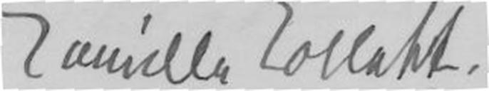Camilla Collett
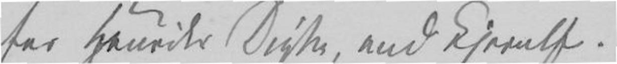for Henriks Digte, end Kjerulf.
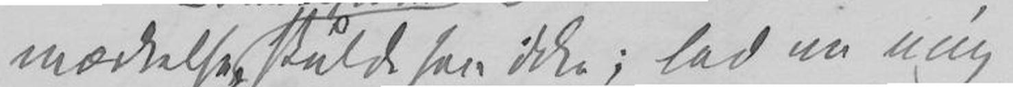mærkelser skulde han ikke; lad nu mig
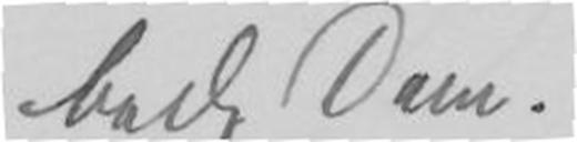bede Dem.
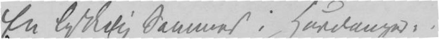En lykkelig Sommer i Hardanger
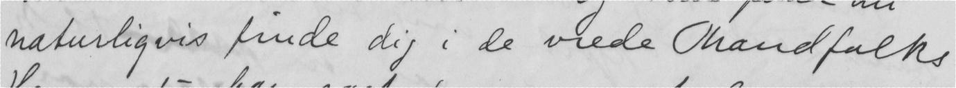naturligvis finde dig i de viede Mandfolks
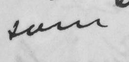som
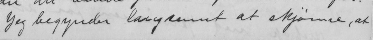Jeg begynder langsemt at skjønne, at
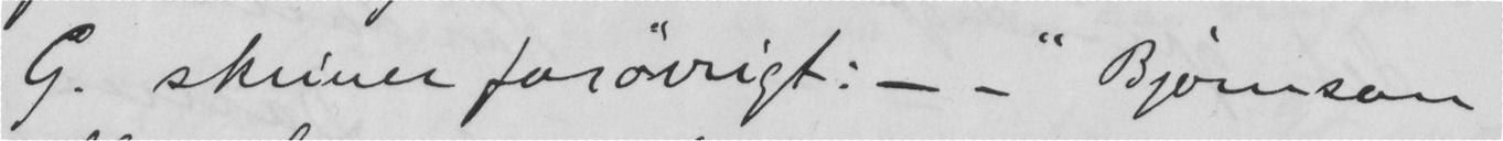G. skriver forøvrigt: - - "Bjørnson
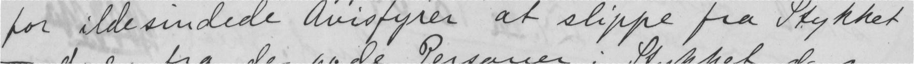for ildesindede Avisfyrer at slippe fra Stykket
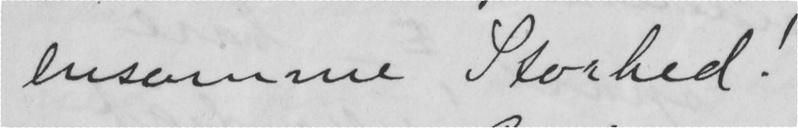ensomme Storhed!
